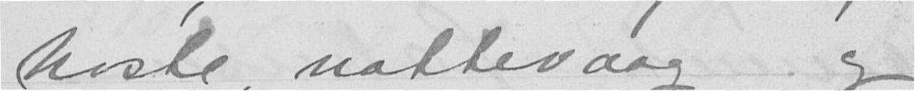hoste, nattevaag og
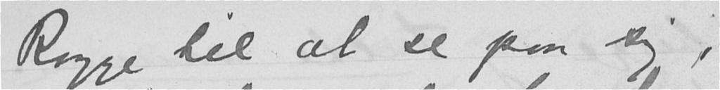Rogge til at se paa sig,
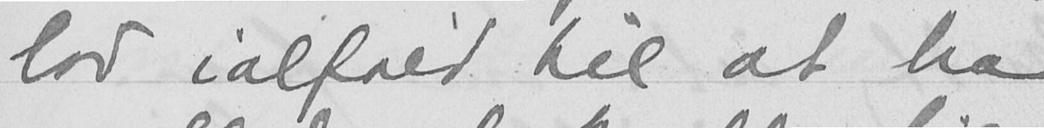lod ialfald til at ha
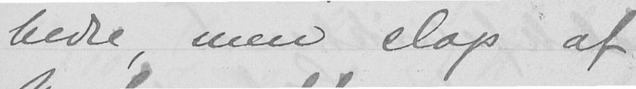bedre, men slap af
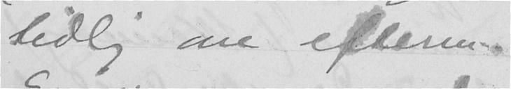tidlig om efterm.
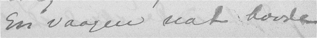En vaagen nat havde
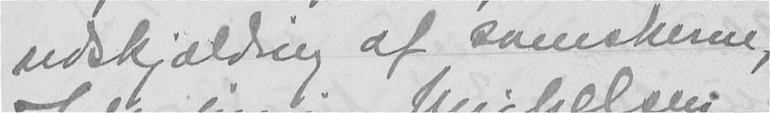udskjælding af svenskerne,
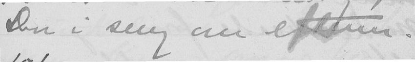Don i seng om efterm.
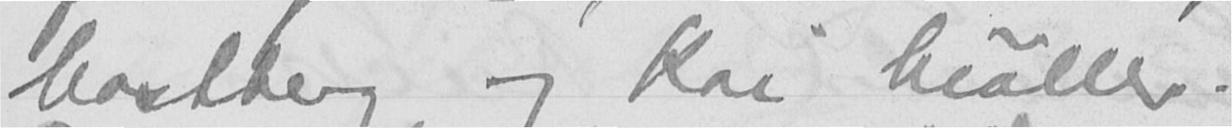Castberg og Kai Møller.
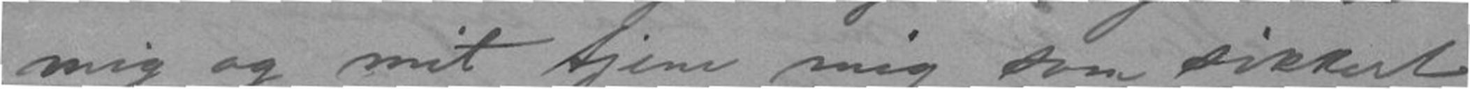mig og mit tjene mig som sikkert
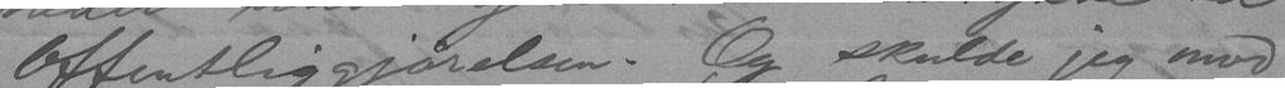Offentliggjørelsen. Og skulde jeg mod
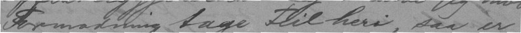Formodning tage Feil heri, saa er
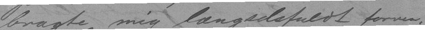bragte mig længselsfuldt forven-
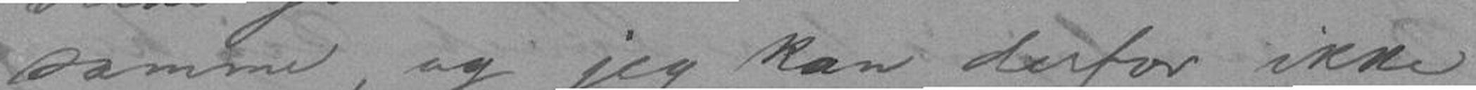samme, og jeg kan derfor ikke
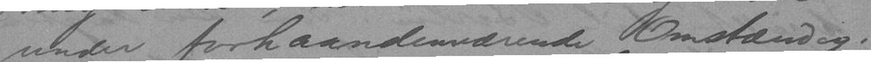under forhaandenværende Omstændig-
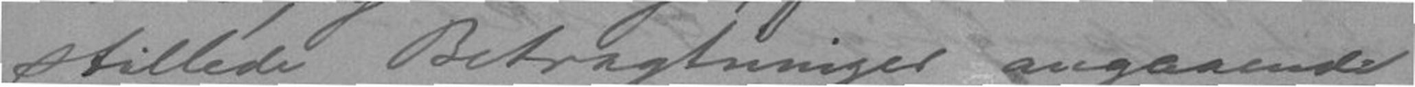stillede Betragtninger angaaende
